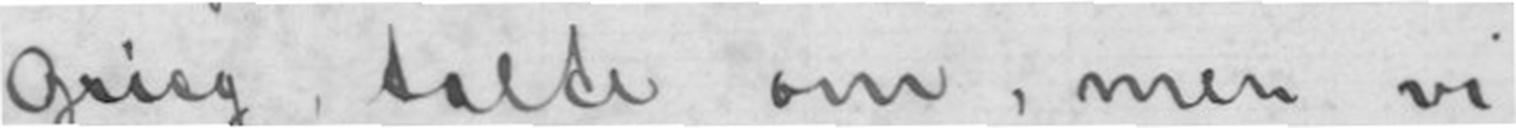Grieg, talte om, men vi
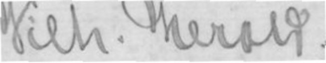Wilh. Herold.
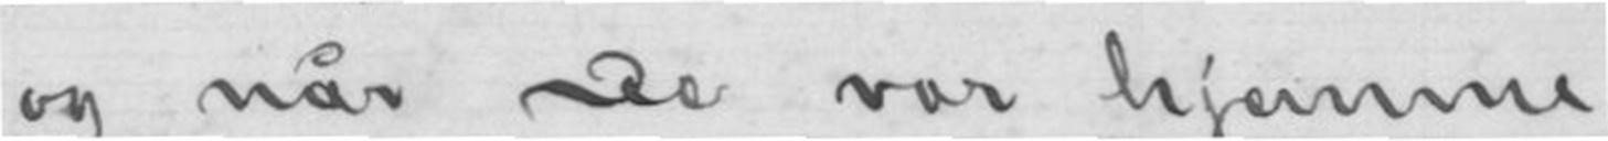og når De var hjemme,
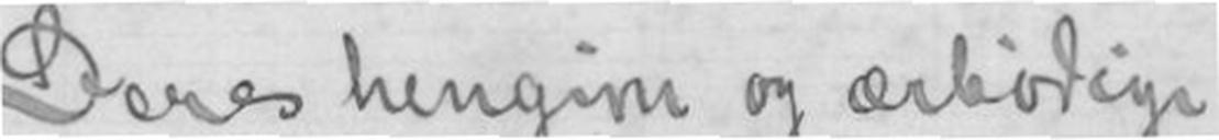Deres hengivne og ærbødige
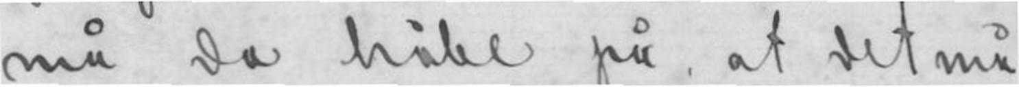må da håbe på, at det må
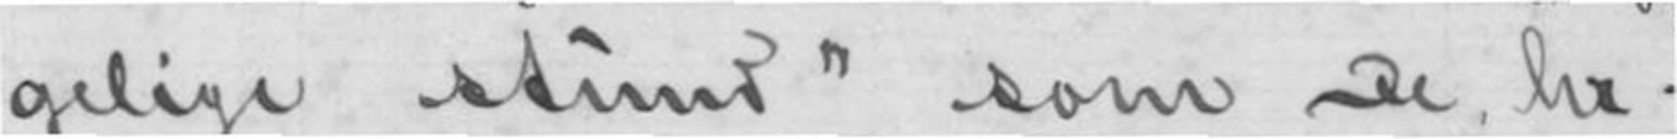gelige stund som De hr.
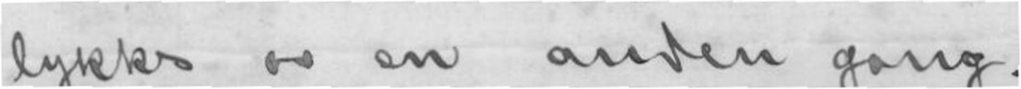lykkes os en anden gang.
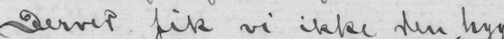Derved fik vi ikke den hyg-
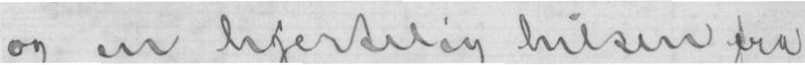og en hjertelig hilsen fra
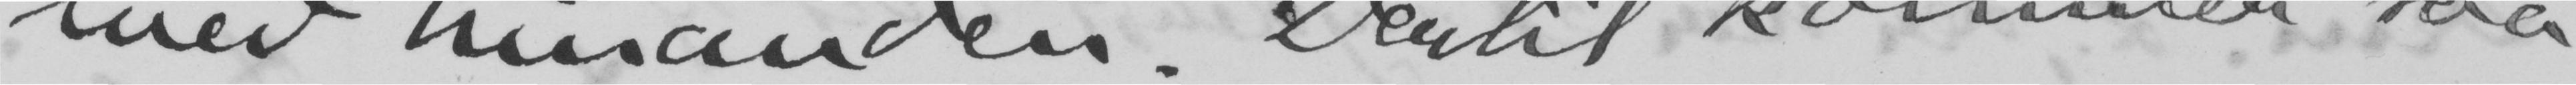med hinanden. Dertil kommer saa
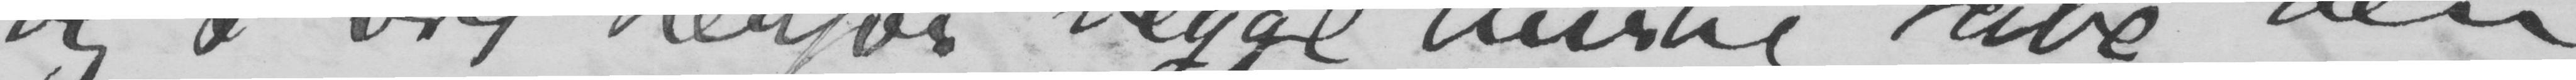og I vil derfor begge hurtig tabe den
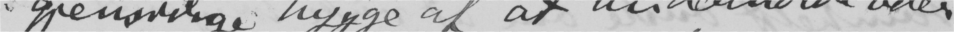gjensidige hygge af at underholde Eder
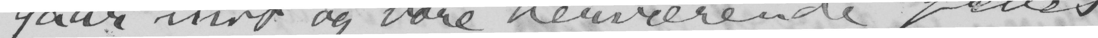gaar mit og vore herværende fælles
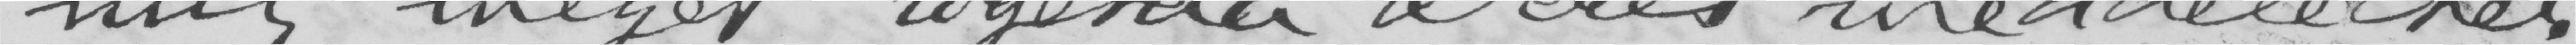mig meget, ligesaa Deres meddelser
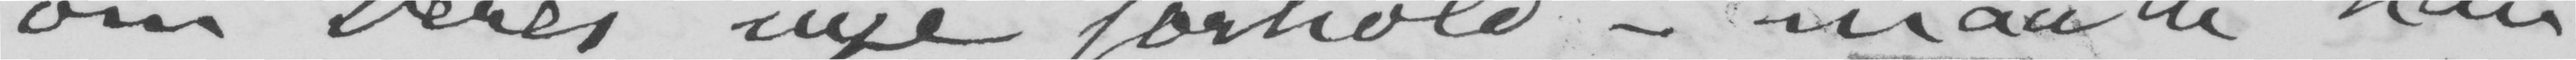om Deres nye forhold, – maatte han
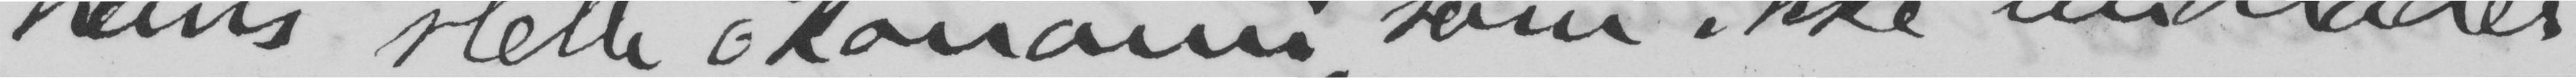hans slette økonomi, som ikke undlader
